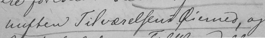nuften Tilværelsens Øiemed, og
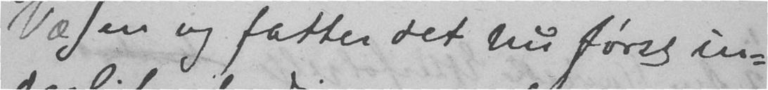Væsen og fatter det nu først in-
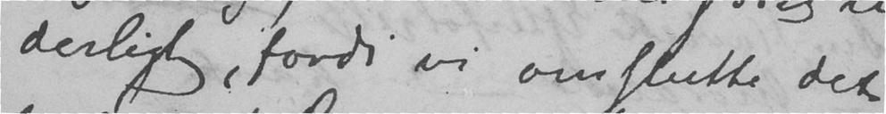derligt, fordi vi omslutte det
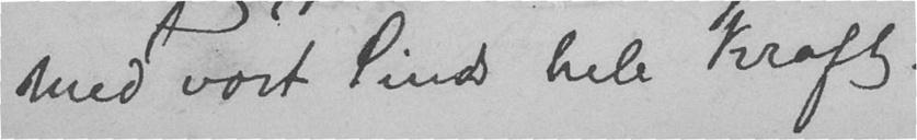med vort Sinds hele Kraft.
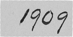1909
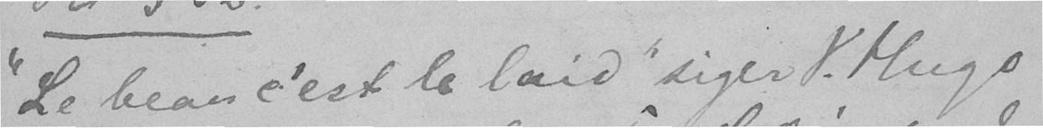"Le beau c'est le laid" siger V. Hugo.
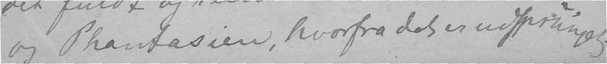og Phantasien, hvorfra det er udsprunget.
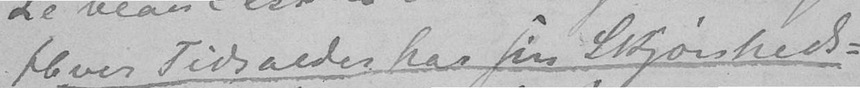Hver Tidsalder har sin Skjønheds-
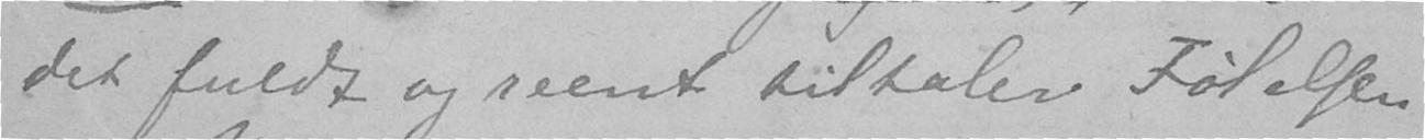det fuldt og reent tiltaler Følelsen
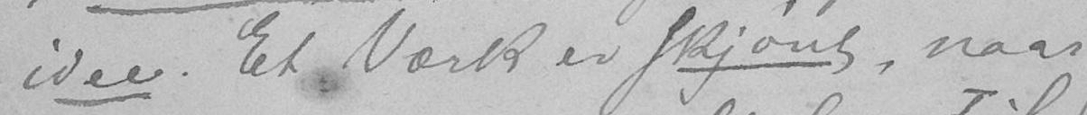idee. Et Værk er skjønt, naar
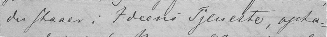der staaer i Ideens Tjeneste, opta-
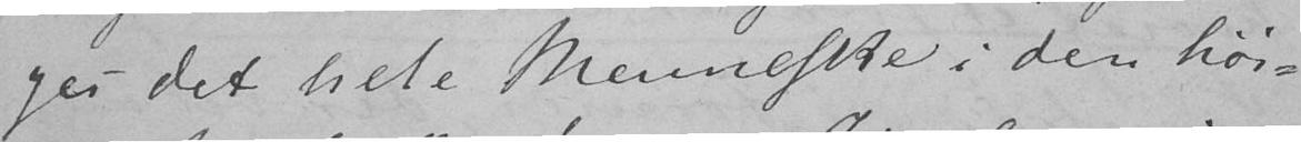ges det hele Menneske i den høi-
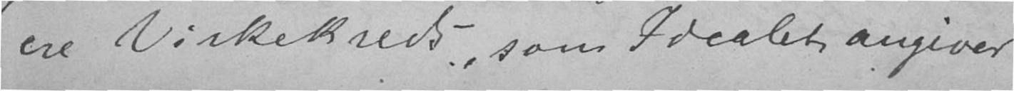ere Virkekreds som Idealet angiver
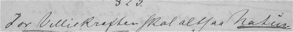For Villiekraften skal altsaa Natur-
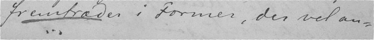fremtræder i Former, der vel an-
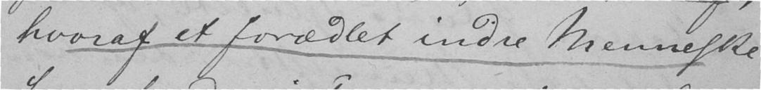hvoraf et forædlet indre Menneske
